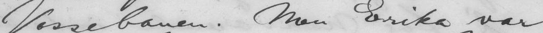Vossebanen. Men Erika var
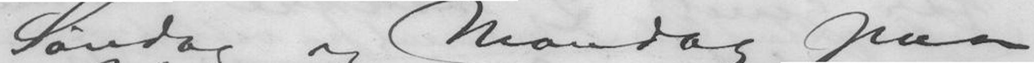Søndag og Mandag paa
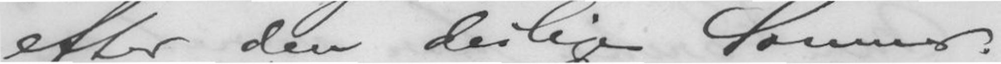efter den deilige Sommer.
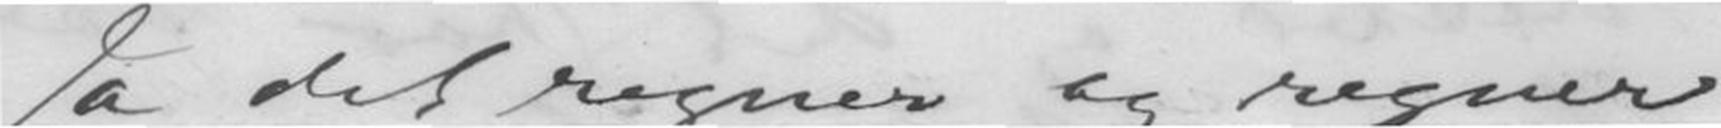Ja det regner og regner
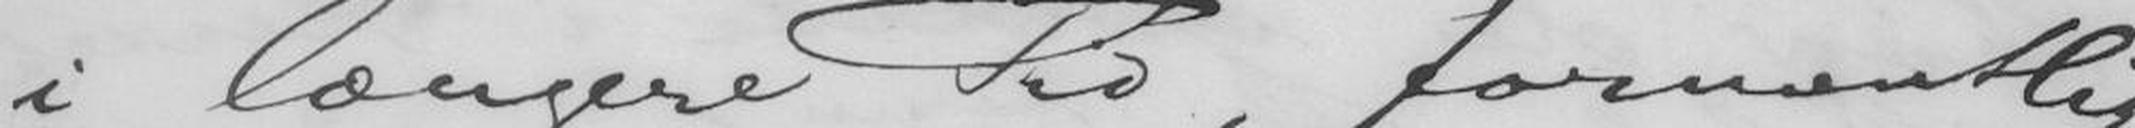i længere Tid, formentlig
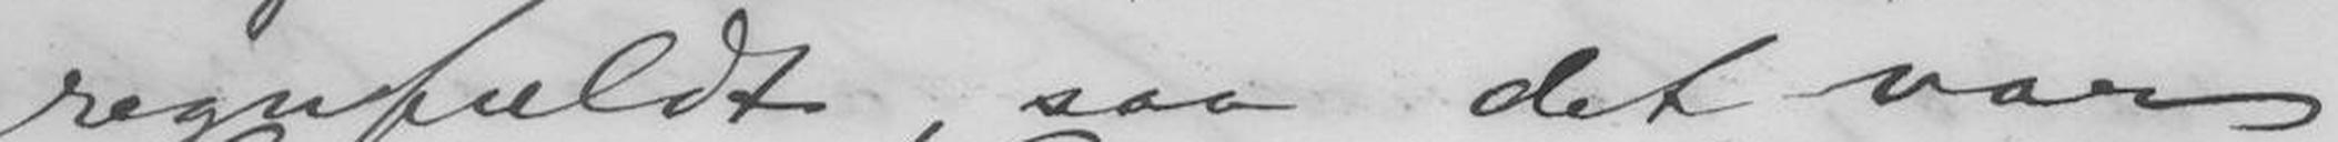regnfuldt, saa det var
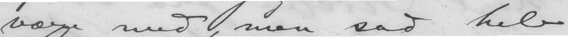være med, men sad hele
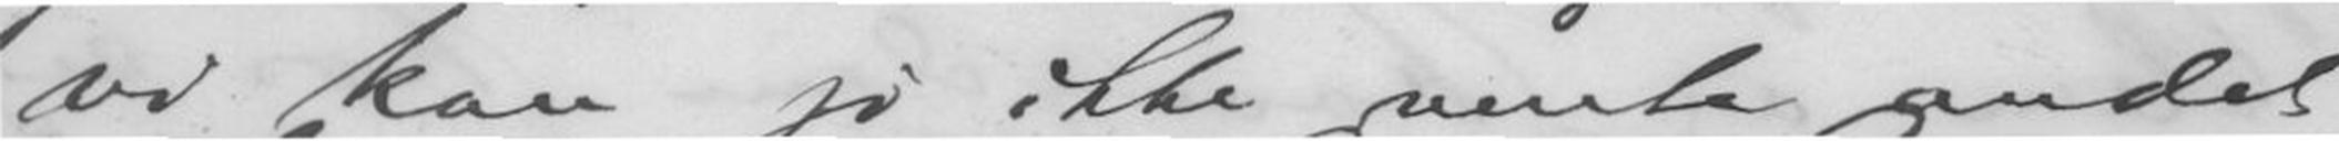vi kan jo ikke vente andet
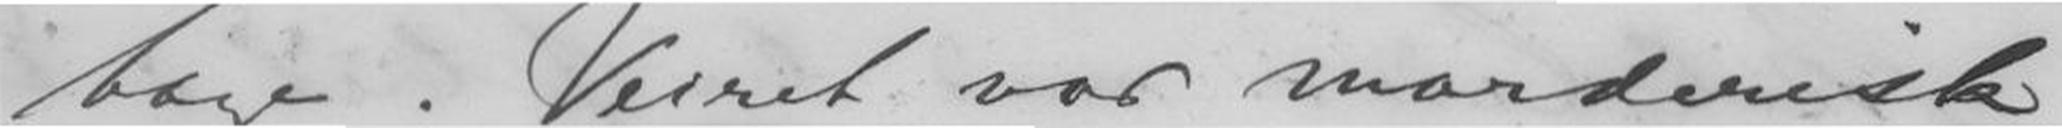bage. Veiret var morderisk
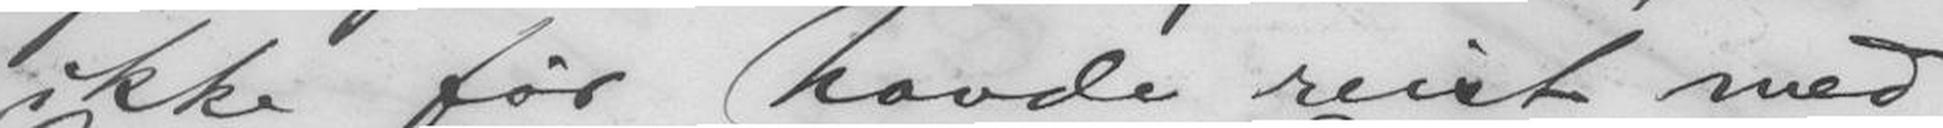ikke før havde reist med
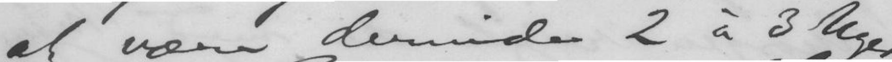at være derinde 2 à 3 Uger
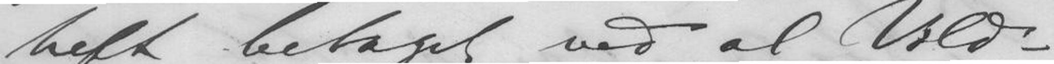helt betaget ved al Vild-
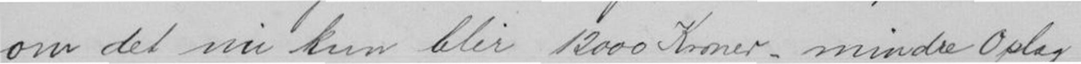om det nu kun blir 12000 Kroner - mindre Oplag
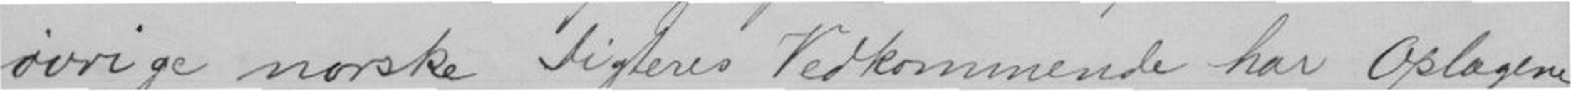øvrige norske Digteres Vedkommende har Oplagene
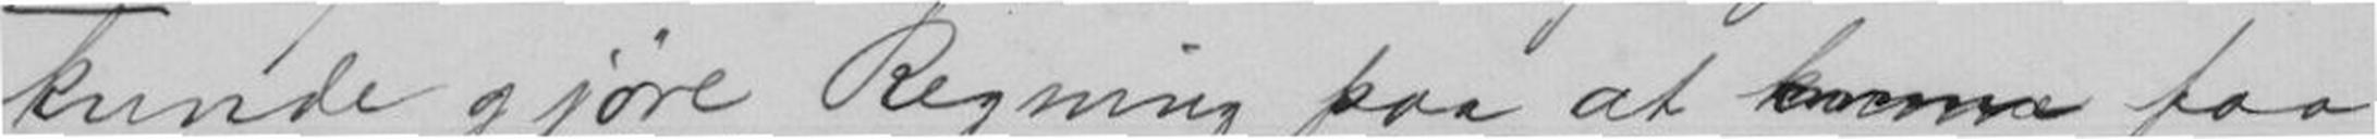kunde gjøre Regning paa at faa
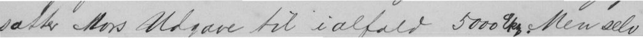sætter Mors Udgave til ialfald 5000 Eks. Men selv
